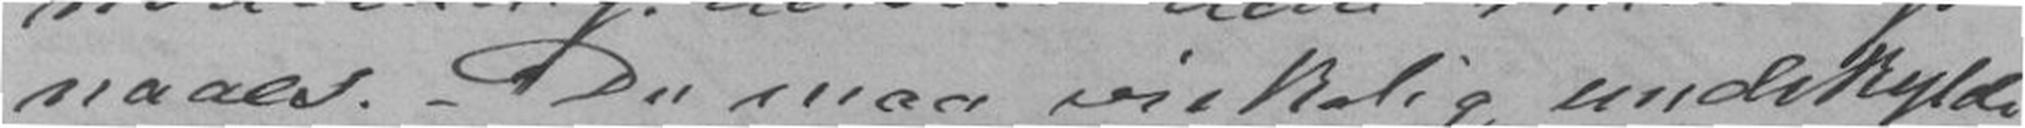naaes. Du maa virkelig undskylde
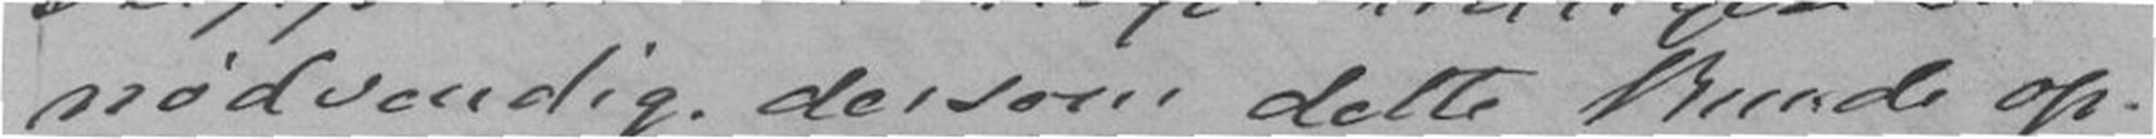nødvendig dersom dette kunde op-
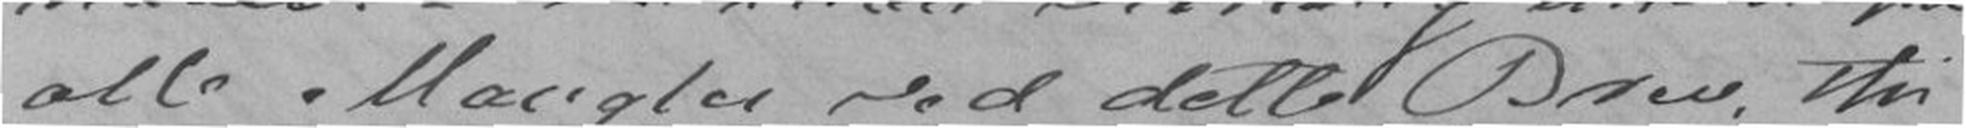alle Mangler ved dette Brev, thi
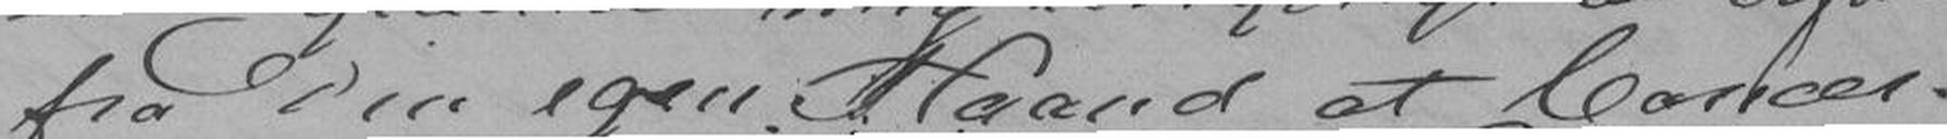fra Din egen Haand at Concer-
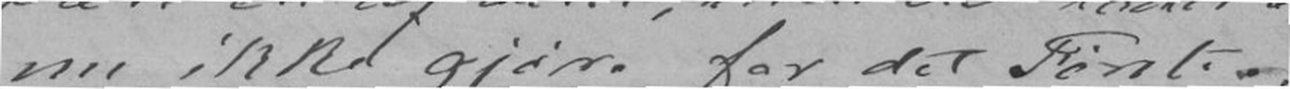nu ikke gjøre for det Første.
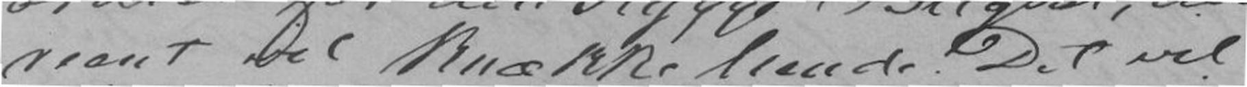reent vil knække hende. Det vil
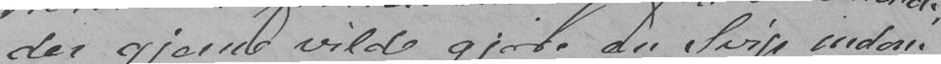der gjerne vilde gjøre en Svip indom
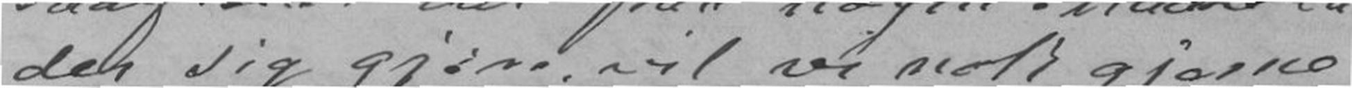der sig gjøre, vil vi nok gjerne
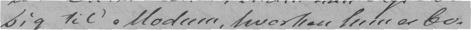sig til Modum, hvorhen hun er be-
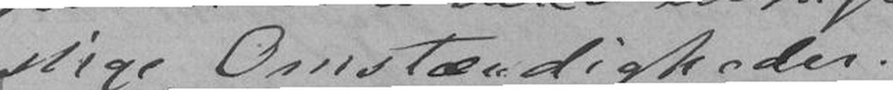slige Omstændigheder.
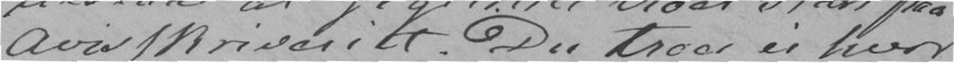Avisskriveriet. Du troer ei hvor
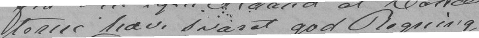terne have svaret god Regning,
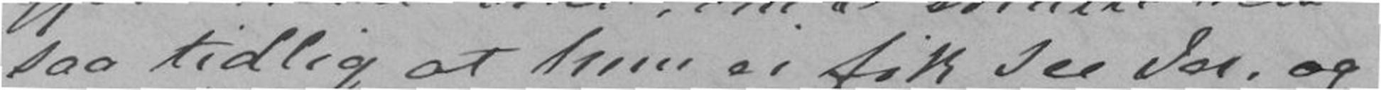saa tidlig at hun ei fik see Jer, og
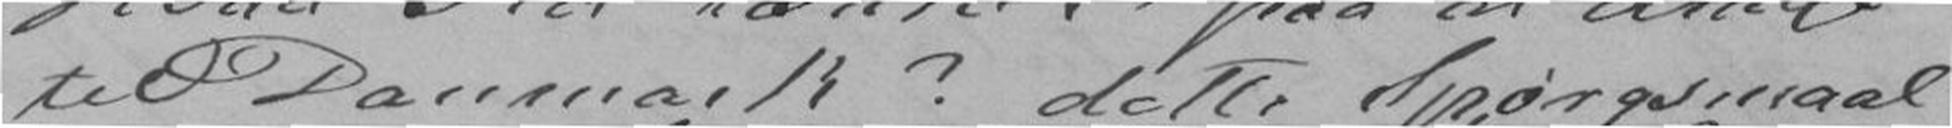til Danmark? dette Spørgsmaal
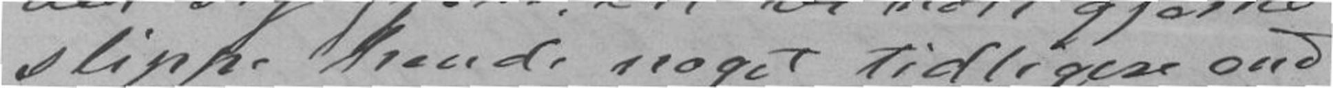slippe hende noget tidligere end
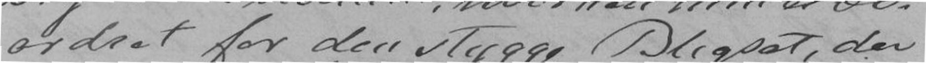ordret for den stygge Blegsot, der
